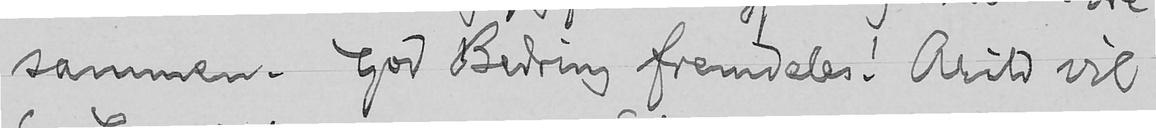sammen. God Bedring fremdeles! Arild vil
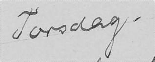Torsdag.
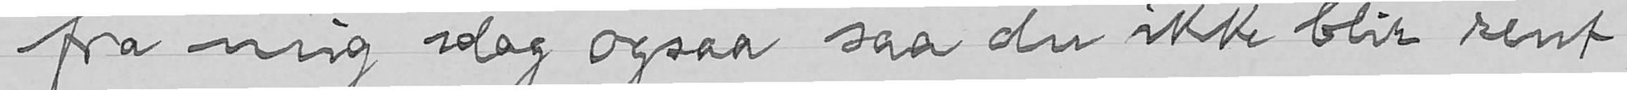fra mig idag ogsaa saa du ikke blir rent
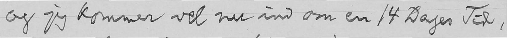og jeg kommer vel nu ind om en 14 Dages Tid,
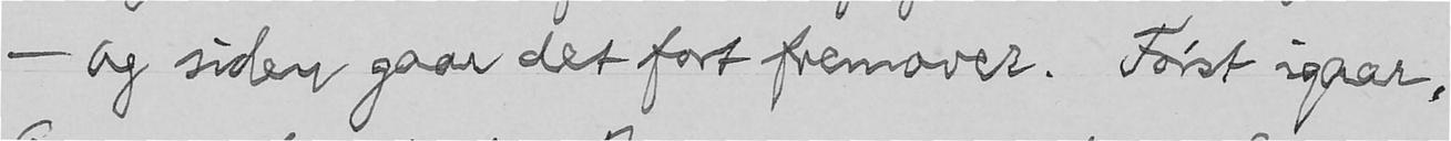- og siden gaar det fort fremover. Først igaar,
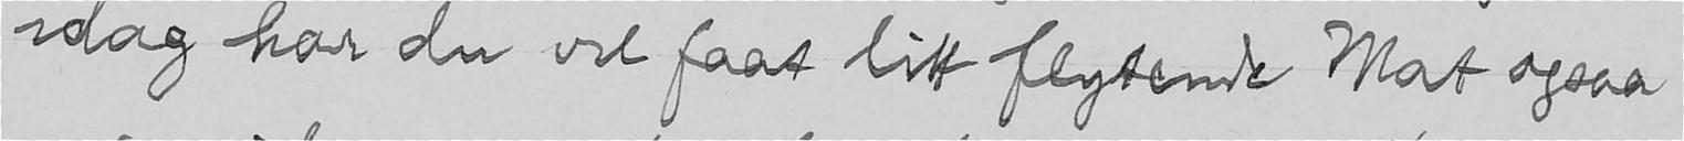idag har du vel faat litt flytende Mat ogsaa
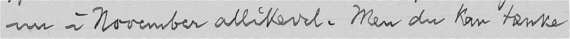nu i November allikevel. Men du kan tænke
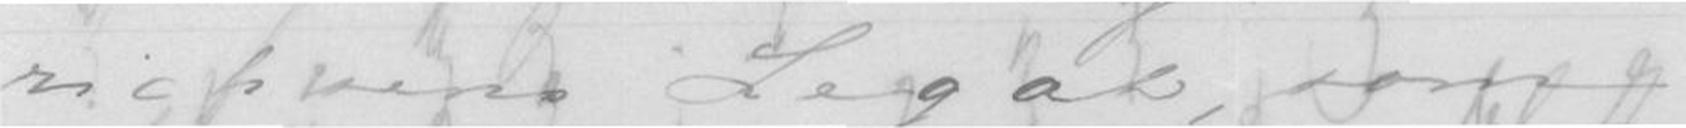richsens Legat, som
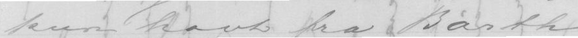kun havt fra Barth
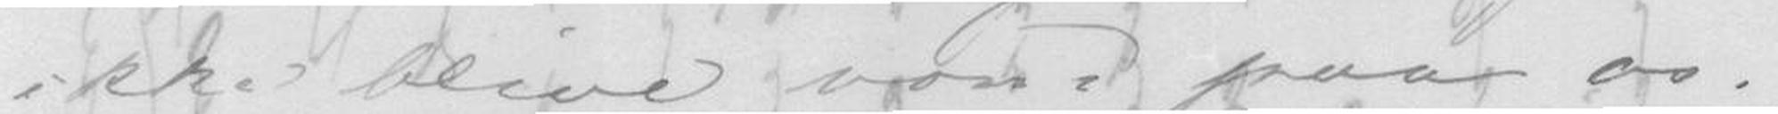ikke blive vond paa os.
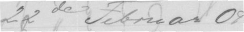22de Februar 07.
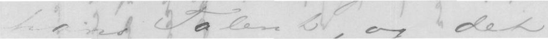hans Talent, og det
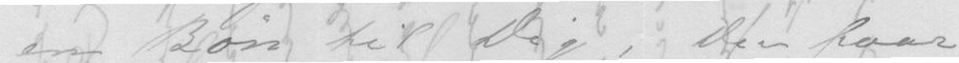en Bøn til Dig, Du faar
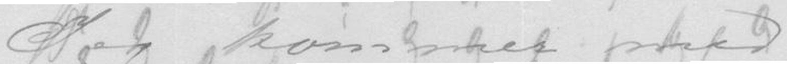Jeg kommer med
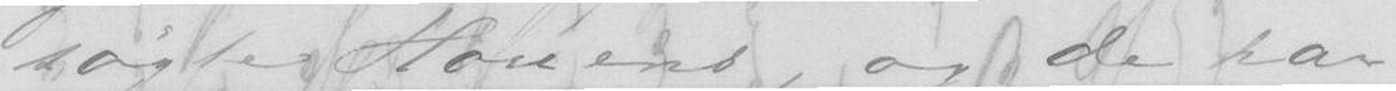søgte Houens, og de har
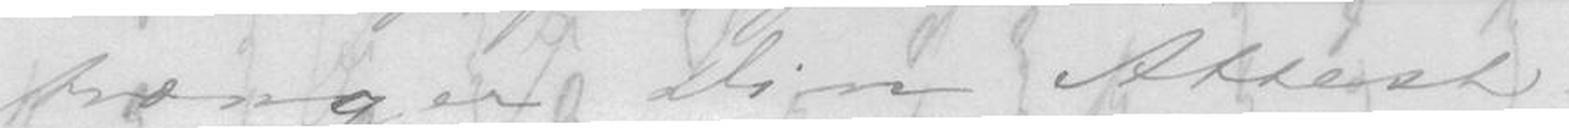trænger Din Attest
